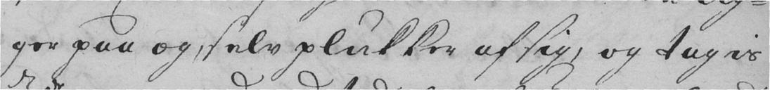ger paa og selv plukker af sig, og tagis
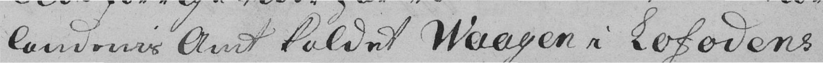landenis Amt kaldet Waagen i Lofodens
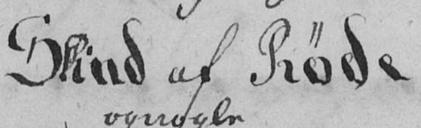Hud af Røde
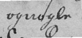og nogle
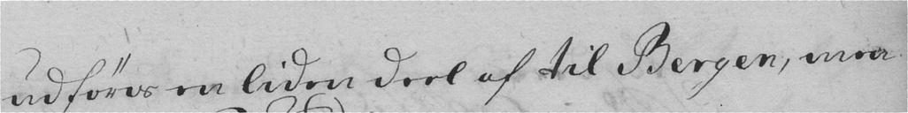udføris en liden deel af til Bergen, men
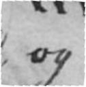og
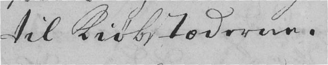til Kiøbstæderne.
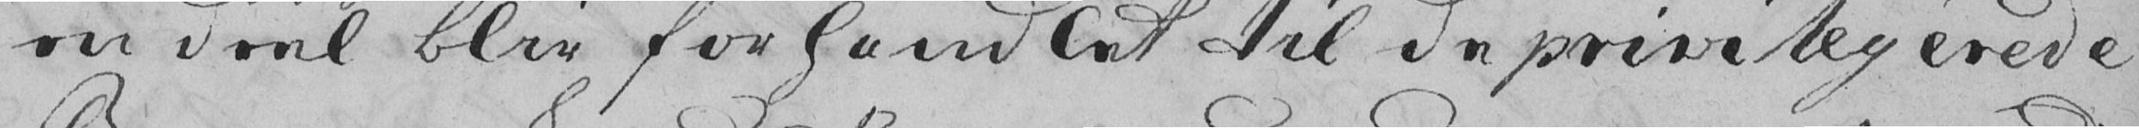en deel blir forhandlet til de priviligerede
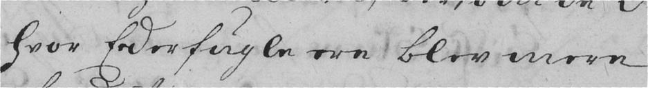hvor Federfuglen ere blev mere
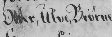Oder, Ulve, Biørne
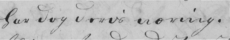har dog deris næring.
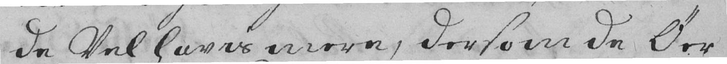de vel havis mere, dersom de Øer
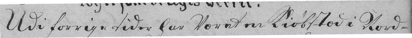Udi forrige tider har Været en Kiøbstad i Nord-
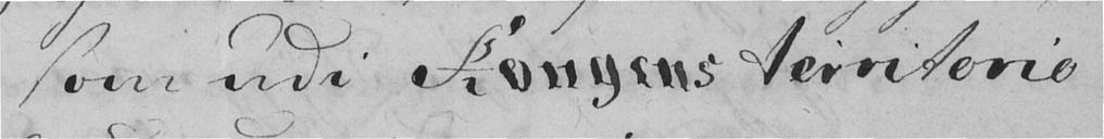som udi Kongens territorio
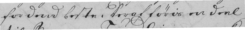for den beste. Deraf føris en deel
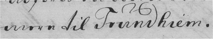mere til Trundhiem.
